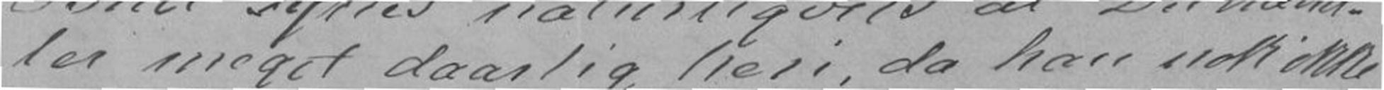ler meget daarlig heri, da han nok ikke
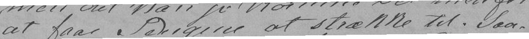at faa Pengene at strække til. Saa-
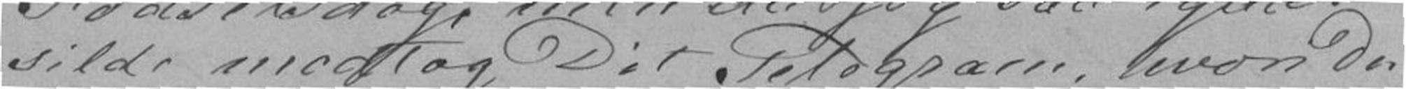silde modtog Dit Telegram, hvor Du
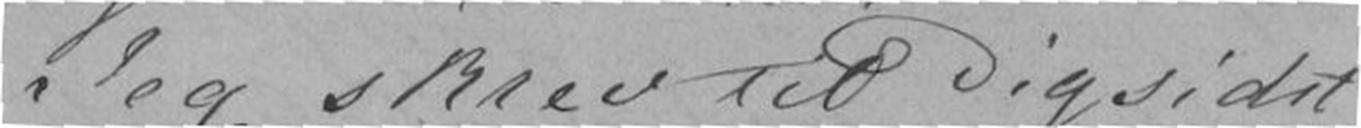Jeg skrev til Dig sidst
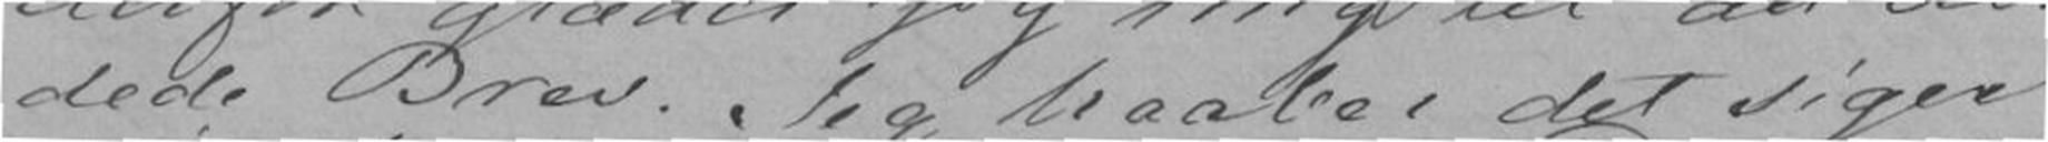dede Brev. Jeg haaber det siger
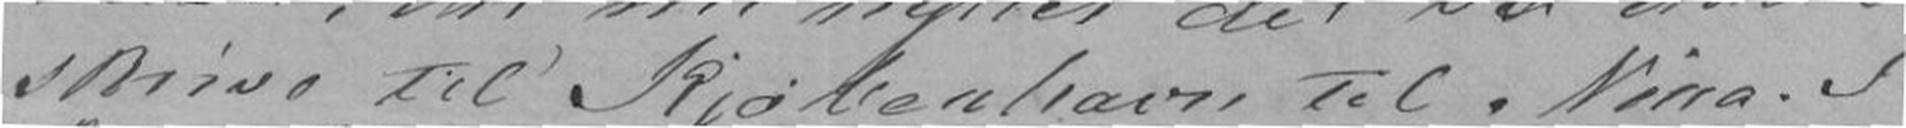skrive til Kjøbenhavn til Nina. I
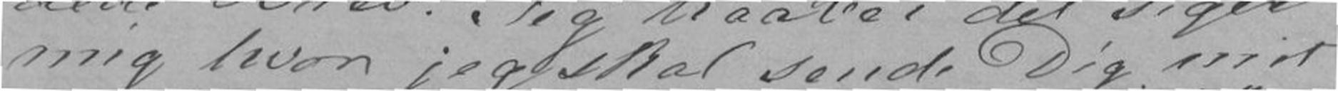mig hvor jeg skal sende Dig mit
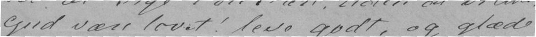Gud være lovet! leve godt, og glæde
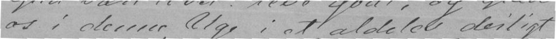os i denne Uge i et aldeles deiligt
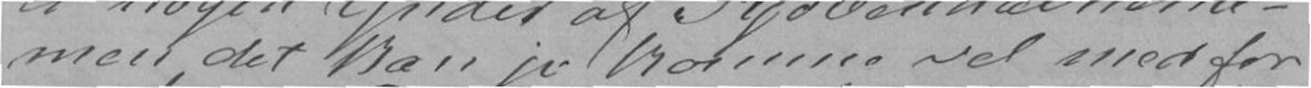men det kan jo komme vel med for
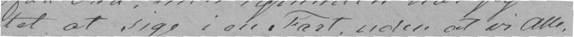tet at sige i en Fart, uden at vi Alle,
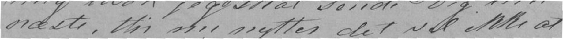næste, thi nu nytter det vel ikke at
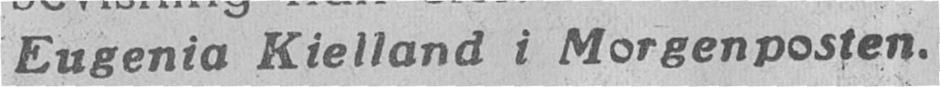Eugenia Kielland i Morgenposten.
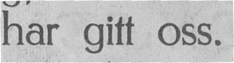har gitt oss.
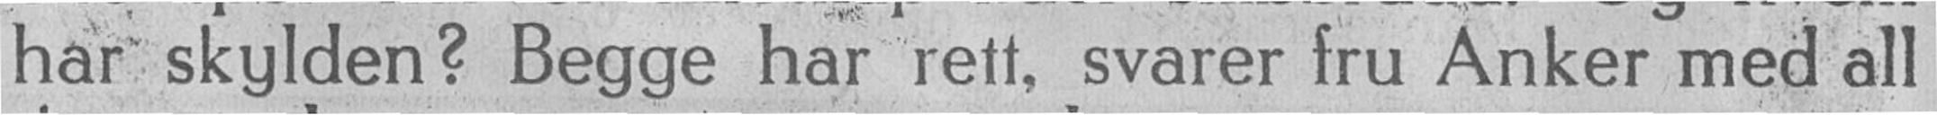har skylden? Begge har rett, svarer fru Anker med all
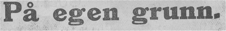På egen grunn.
